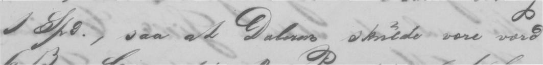1 Spd., saa at Daleren skulde være værd
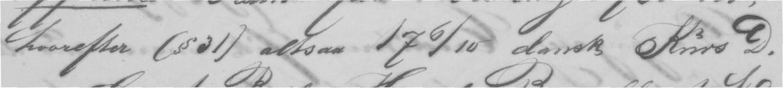hvorefter (§ 31) altsaa 17⁶⁄₁₀ dansk Kurs D.
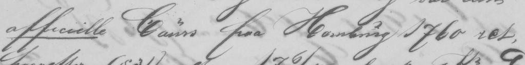officielle Cours paa Hamburg 1760 ret,
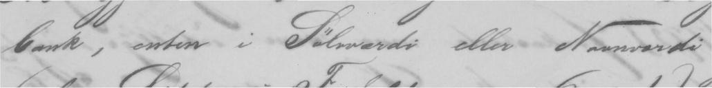bank, enten i Sølvværdi eller Navnværdi
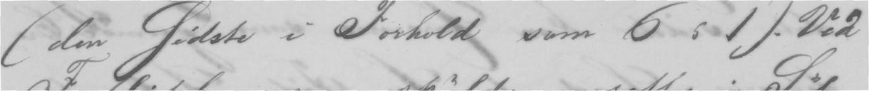(den sidste i Forhold som 6 : 1). Ved
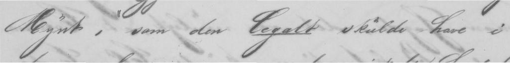Mynt, som den legalt skulde have i
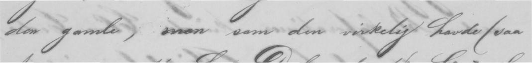den gamle, men som den virkelig havde (saa
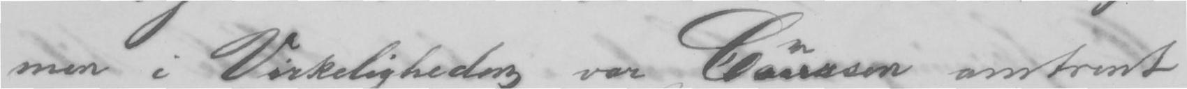men i Virkeligheden var Coursen omtrent
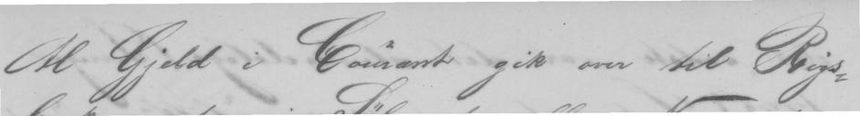Al Gjeld i Courant gik over til Rigs-
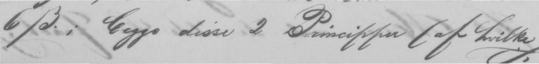6 ẞ; begge disse 2 Principper (af hvilke
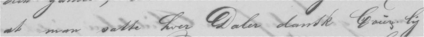at man satte hver Daler dansk Courz. lig
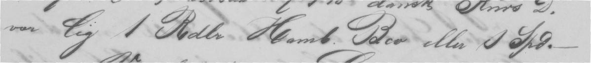var lig 1. Rdlr Hamb. Bev eller 1 Spd.
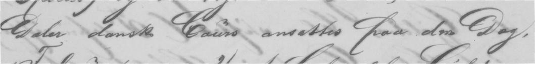Daler dansk Cours omsattes paa den Dag.
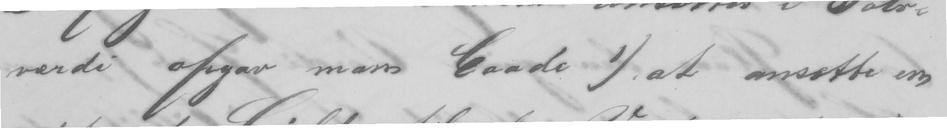værdi opgav man baade 1) at omsætte en
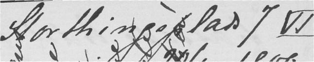Storthingsplads 7 VI
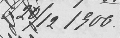20/12 1900.
